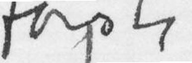første
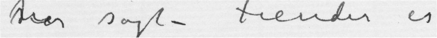har sagt – Fienden er
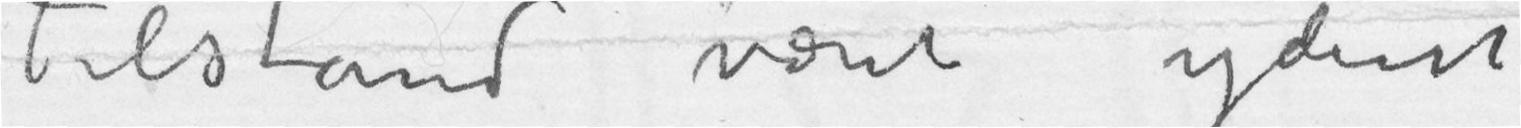Tilstand været yderst
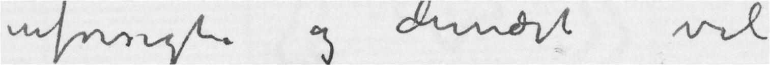uforsigti og dernæst vil
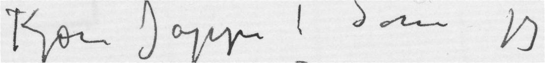Kjære Jappe! Som jeg
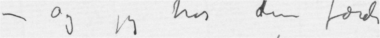– Og jeg tror dem færdi
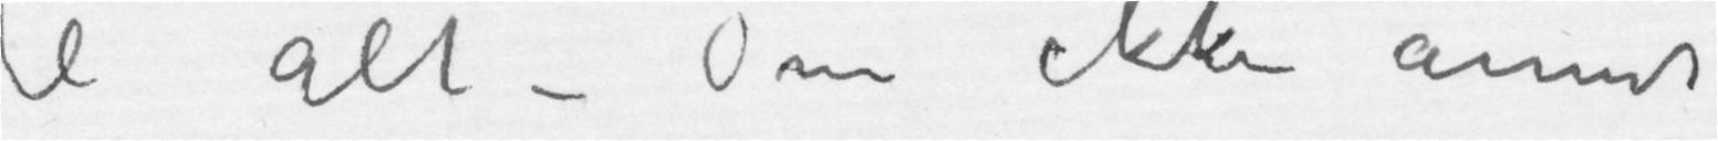til alt – Om ikke annet
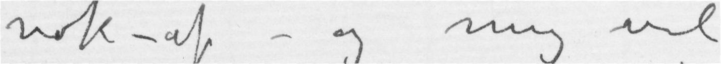nok – af – og mig vil
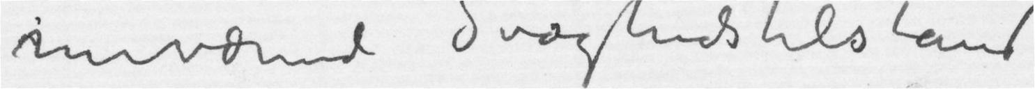nuværende Svaghedstilstand
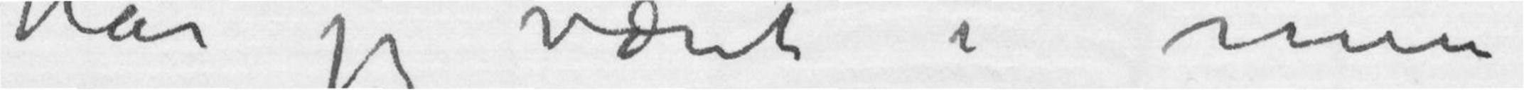har jeg været i mine
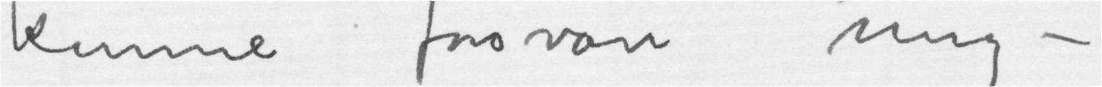kunne forsvare mig –
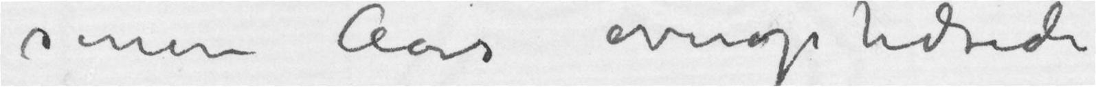senere Aars overophidsede
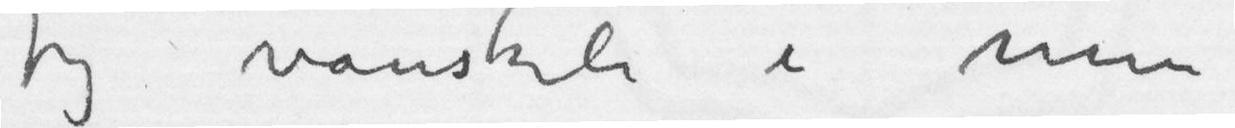jeg vanskeli i min
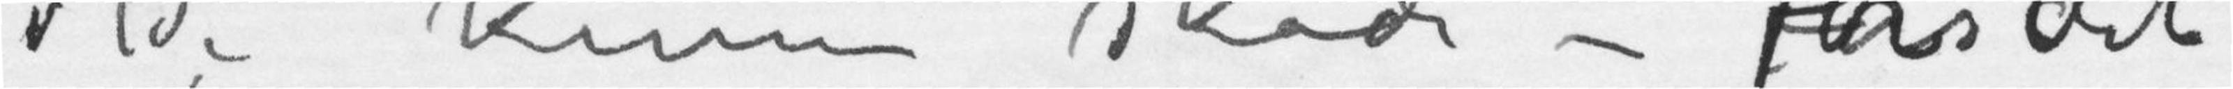De kunne skade – For det
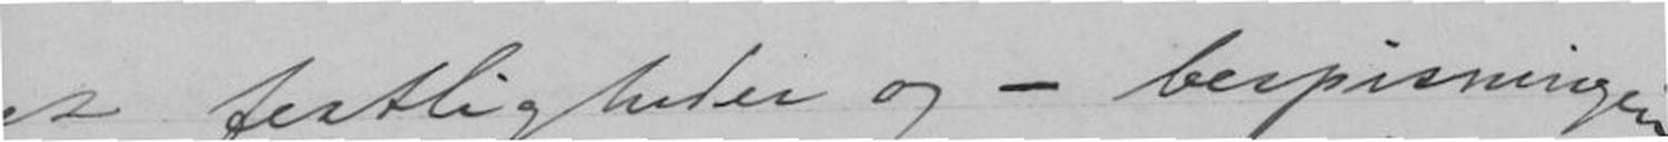festligheder og - bespisningen.
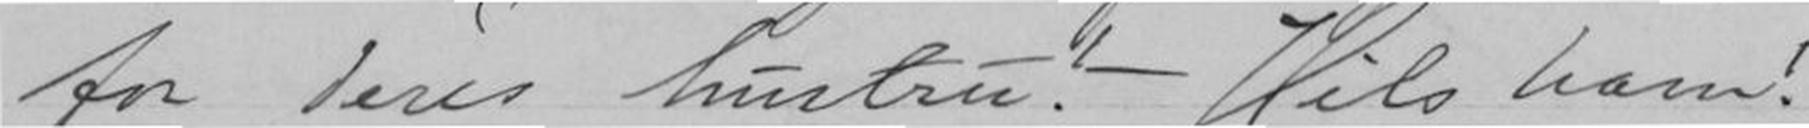for deres hustru! - Hils ham!
